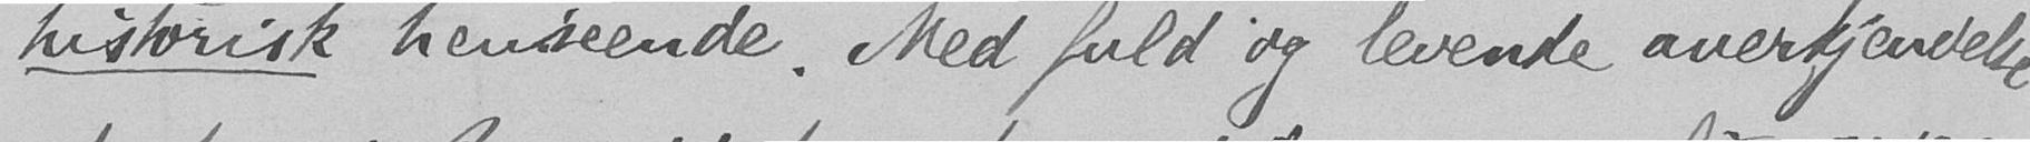historisk henseende. Med fuld og levende anerkjendelse
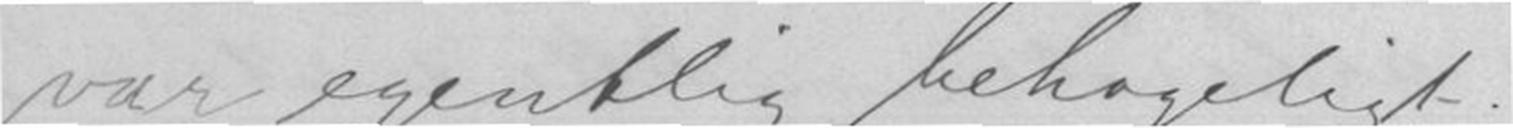var egentlig behageligt
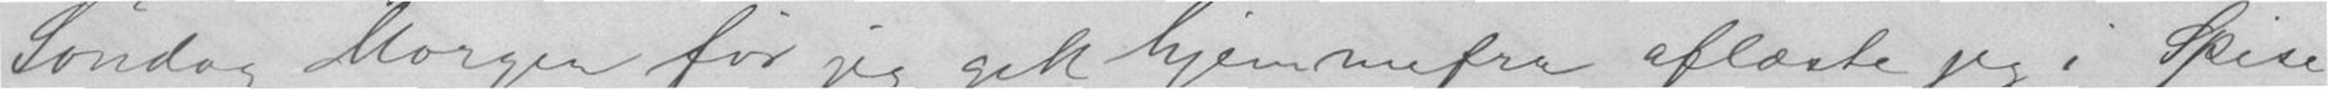Søndag Morgen før jeg gik hjemmefra aflæste jeg i Spise-
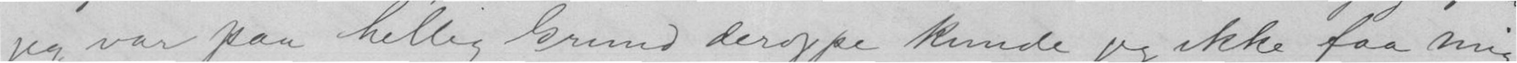jeg var paa hellig Grund deroppe kunde jeg ikke faa mig
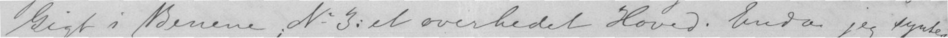Gikt i Benene, N.3: et overhedet Hoved. Enda jeg syntes
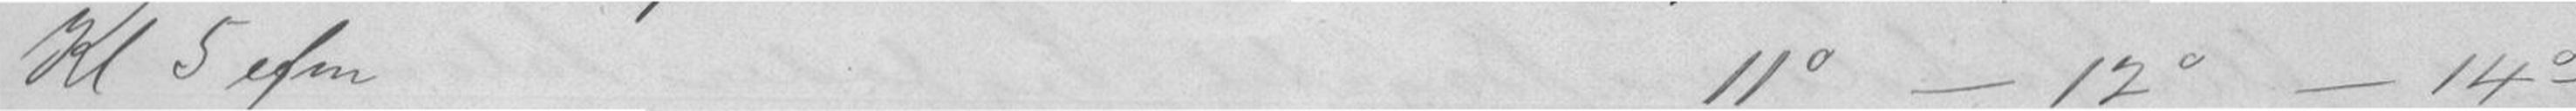Kl 5 efm.11° - 12° - 14°
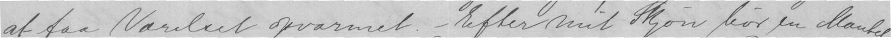at faa Værelset opvarmet. - Efter mit Skjøn bør en Mantel
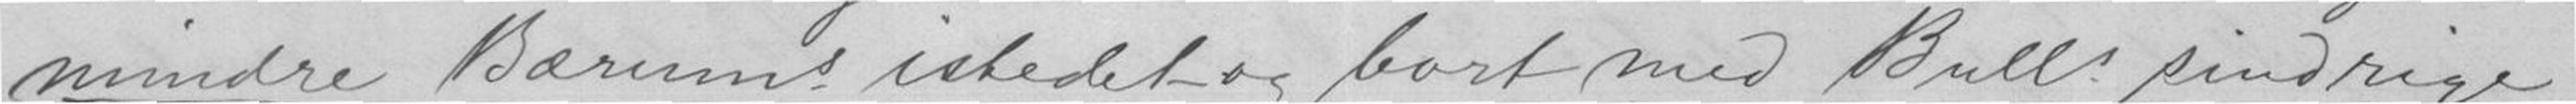mindre Bærums istedet og bort med Bulls sindrige
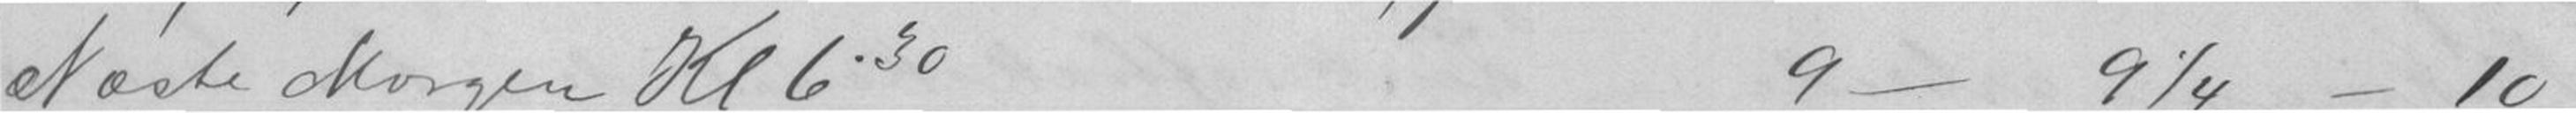Næste Morgen Kl 6.30 9 - 9¼ - 10
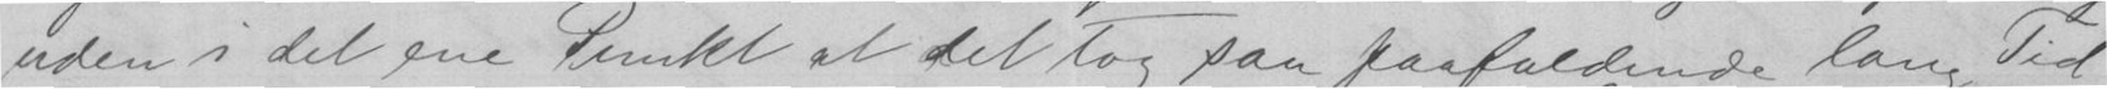uden i det ene Punkt at det tog saa paafaldende lang Tid
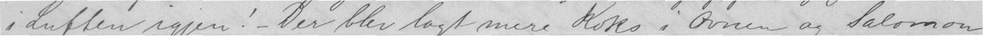i Luften igjen! - Der blev lagt mere Koks i Ovnen og Salomon
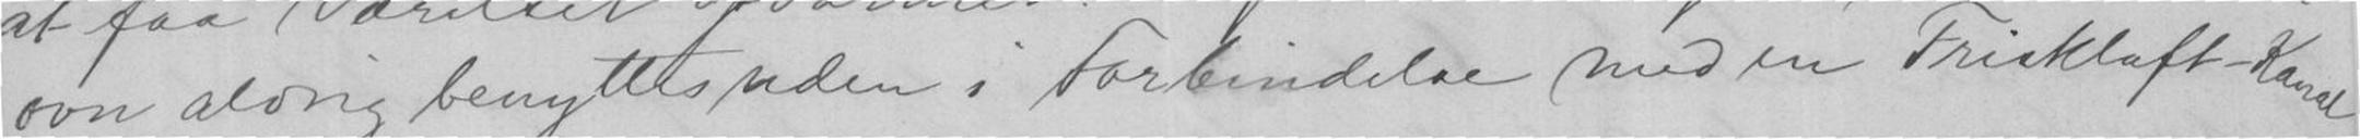ovn aldrig benyttes uden i Forbindelse med en Friskluft-Kanal
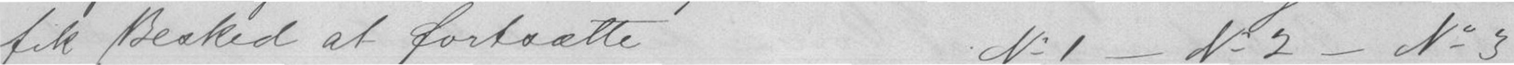fik Besked at fortsætteN.1- N.2 - N.3
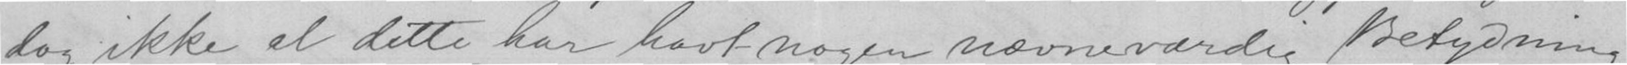dog ikke at dette har havt nogen nævneværdig Betydning
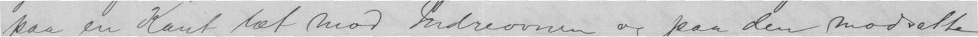paa en Kant tæt mod Indreovnen og paa den modsatte
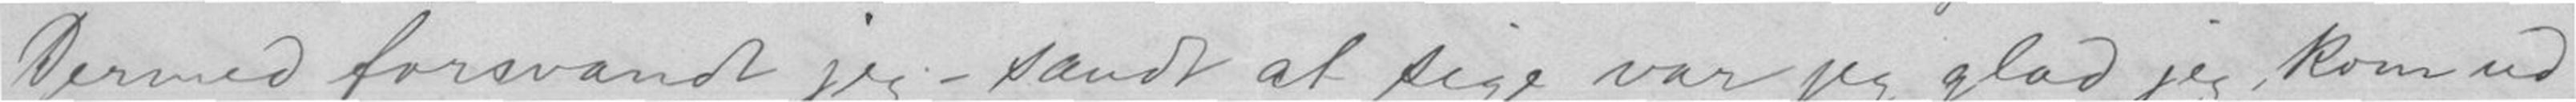Dermed forsvandt jeg - sandt at sige var jeg glad jeg kom ud
